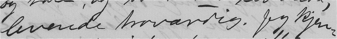levende troværdig. Jeg kjen-
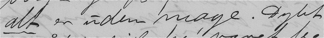alt er uden mage. Dybt
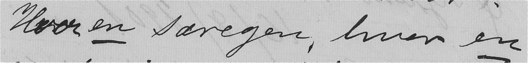Hver en særegen, hver en
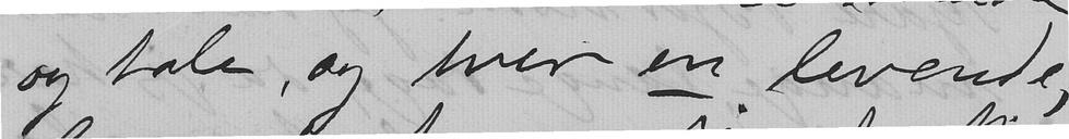og tale, og hver en levende,
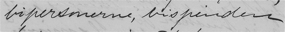bipersonerne, bispinden
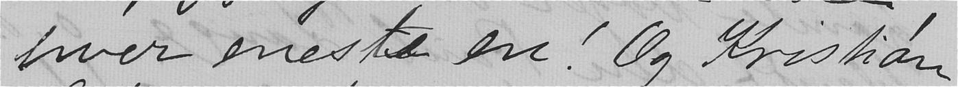hver eneste en! Og Kristian
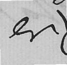er
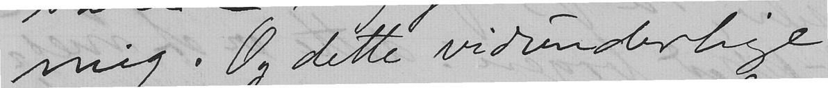mig. Og dette vidunderlige
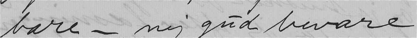bare - nej gud bevare
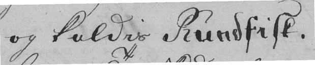og kaldis Rundfisk.
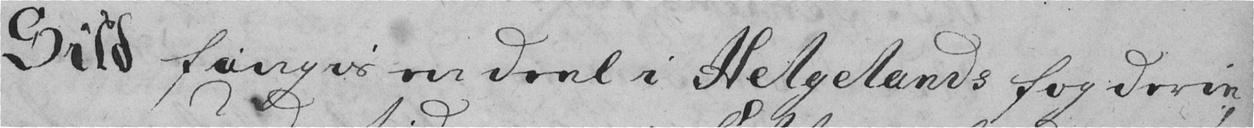Sild fangis en deel i Helgelands fogderie,
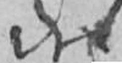det
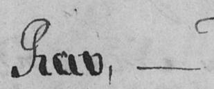Rav, -
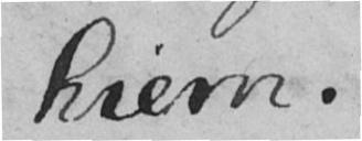hiem.
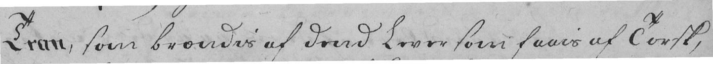Tran, som brændis af dend Lever som faais af Torsk,
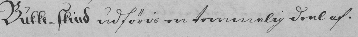Bukke-skind udføres en temmelig deel af.
